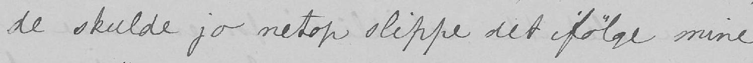de skulde jo netop slippe det ifølge mine
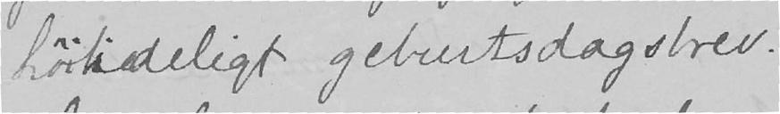høitideligt geburtsdagsbrev.
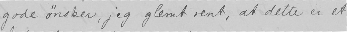gode ønsker, jeg glemt rent, at dette er et
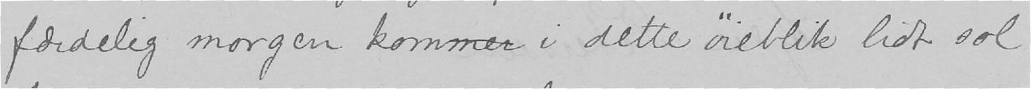færdelig morgen kommer i dette øieblik lidt sol
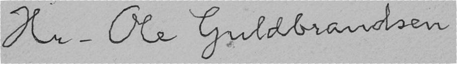Hr. Ole Guldbrandsen
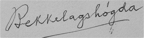Bekkelagshøgda
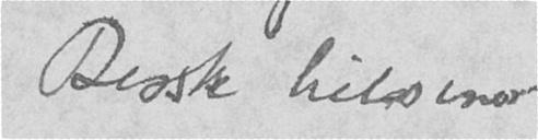Beste hilsener
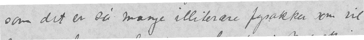som det er så mange illiterære fysakker som vil
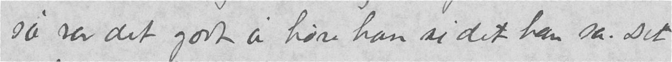så var det godt av høre han si det han sa. Det
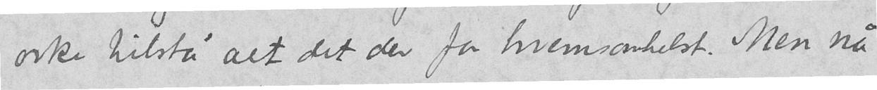orke tilstå alt det der for hvemsomhelst. Men nå
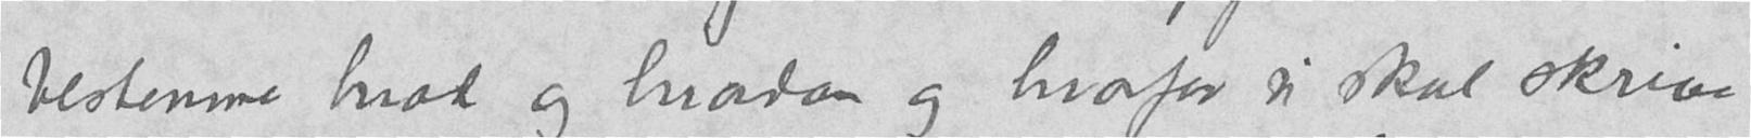bestemme hvad og hvordan og hvorfor vi skal skrive
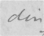din
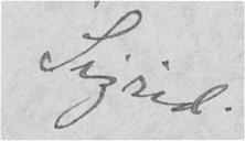Sigrid.
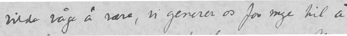vilde våge å være, vi generer os for mye til å
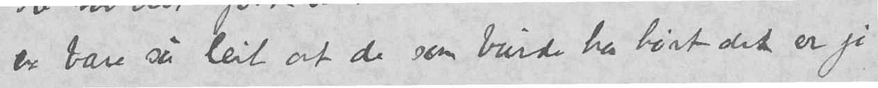er bare så leit at de som burde ha hørt det er jo
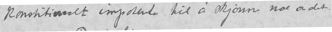konstitionelt impotente til å skjønne noe av det.
